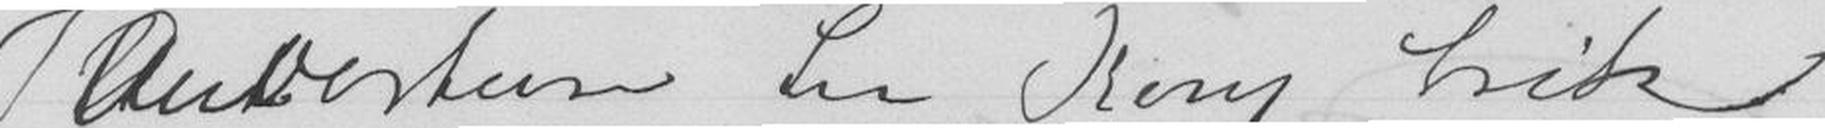Ouverture til Kong Erik
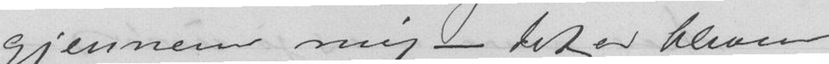gjennem mig - det er bleven
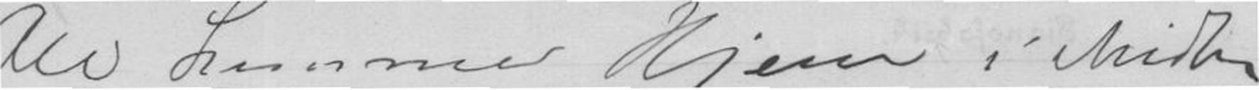Ole kommer Hjem i Midten
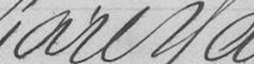Karl Hals
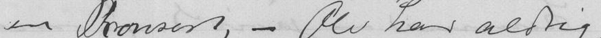en Konsert, - Ole har aldrig
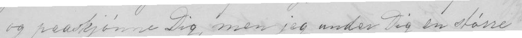og paaskjønne Dig, men jeg under Dig en større
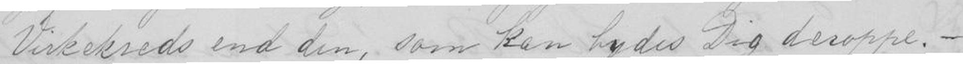Virkekreds end den, som kan Lydes Dig deroppe.-
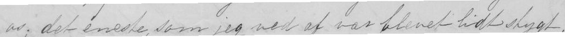os; det eneste, som jeg ved af vor blevet Lidt stygt,
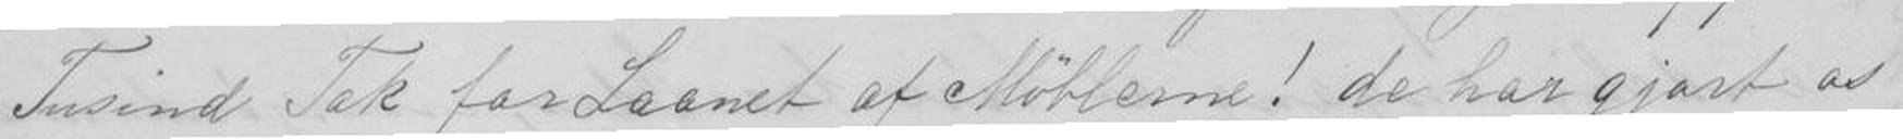Tusind Tak for Laanet af Møblene! de har gjort os
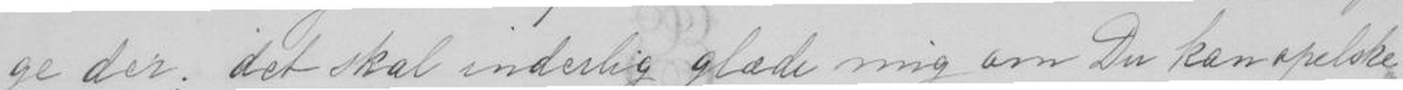ge der; det skal inderlig glæde mig om Du kan opelske
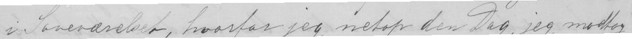i soveværelset, hvorfor jeg netop den Dag, modtog
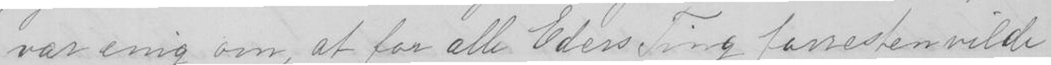var enig om, at for alle Eders Ting forresten vilde
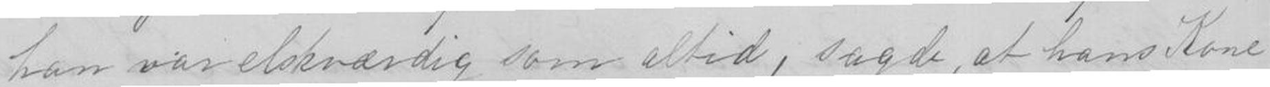han var elskværdig som altid, sagde, at hans Kone
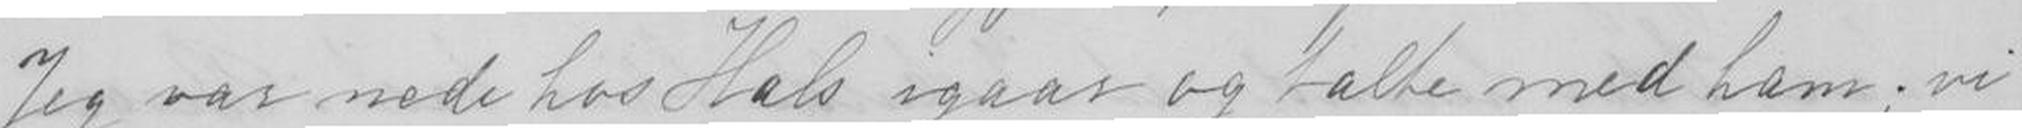Jeg var nede hos Hals igaar og talte med ham; vi
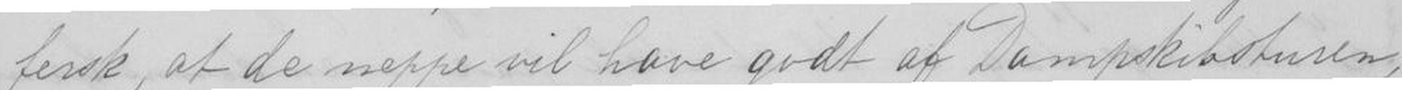fersk, at de neppe vil have godt af Dampskibsturen,
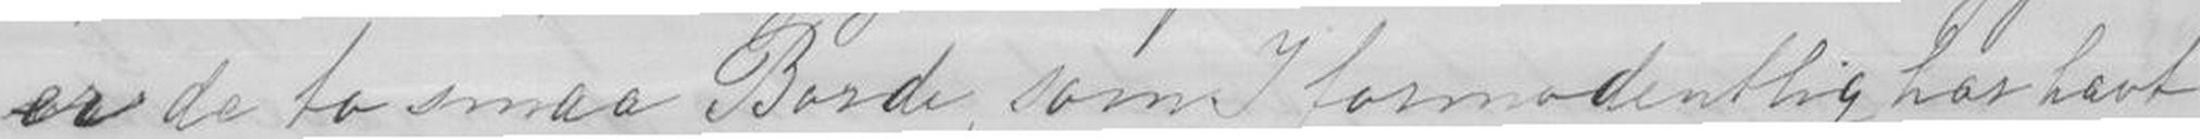er de to smaa Borde, som I formodentlig har lavt
